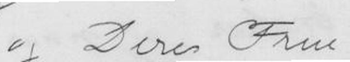og Deres Frue
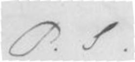P.S.
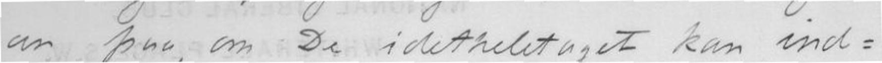an paa, om De idetheletaget kan ind-
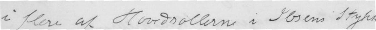i flere af Hovedrollerne i Ibsens Stykker
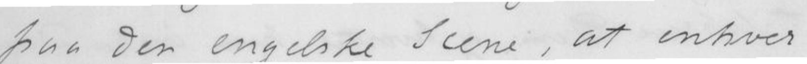paa den engelske Scene, at enhver
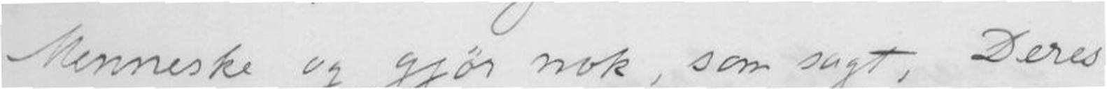Menneske og gjør nok, som sagt, Deres
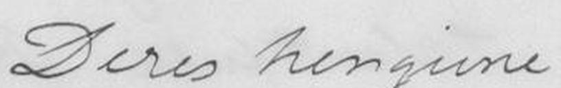Deres hengivne
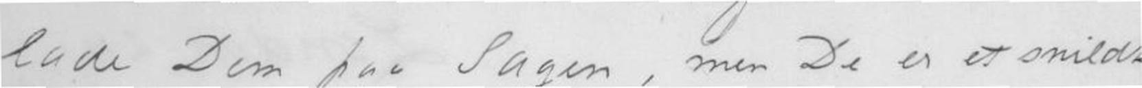lade Dem paa Sagen, men De er et snildt
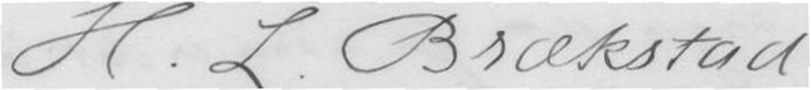H. L. Brækstad
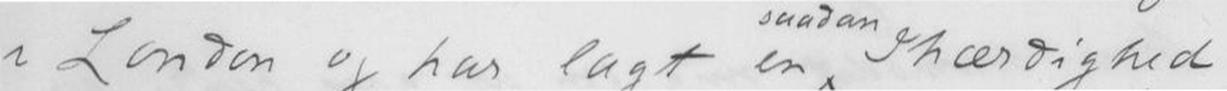i London og har lagt en Ihærdighed
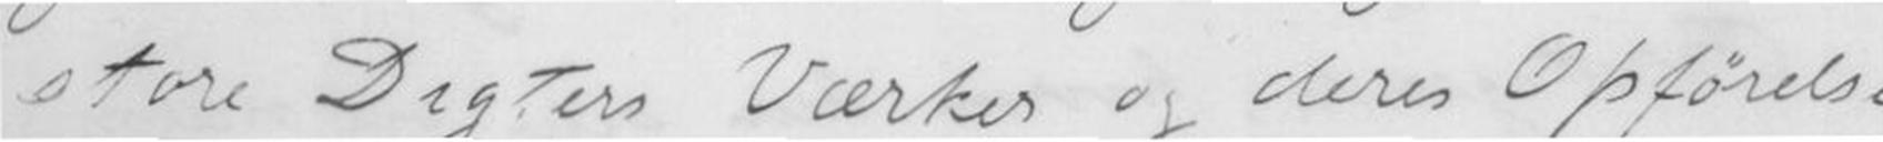store Digters Værker og deres Opførelse
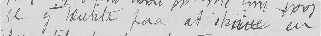ge og tænkte paa at skrive en
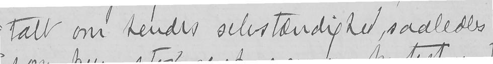talt om hendes selvstændighed, saaledes
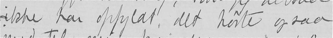ikke har oppyldt, det hørte ogsaa
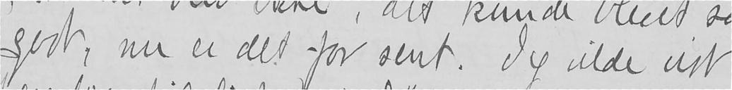godt, nu er det for sent. Jeg vilde vist
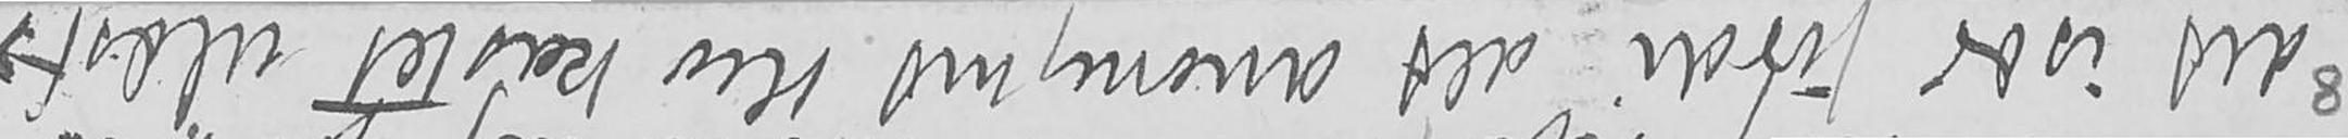det især fordi alt anonymt blev kastet ulæst
